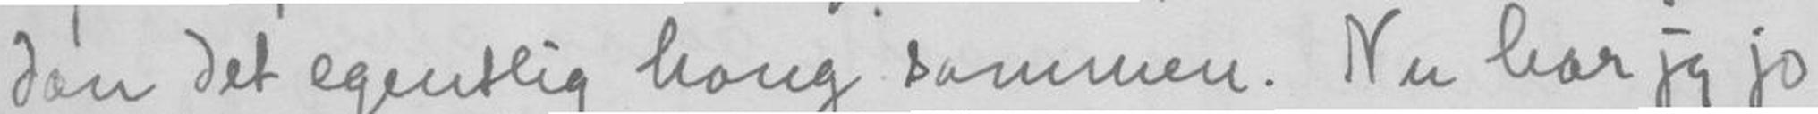dan det egentlig hang sammen, Nu har jeg jo
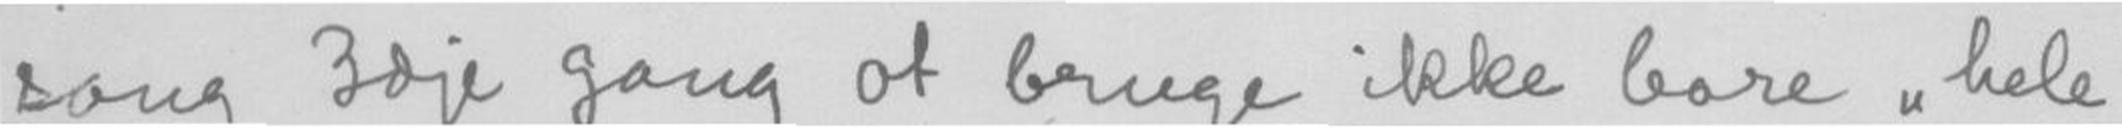sang 3dje gang at bruge ikke bare hele
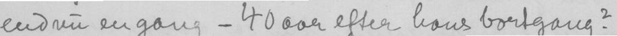endnu engang - 40 aar efter hans bortgang?
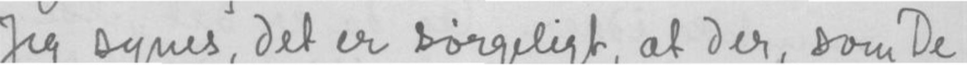Jeg synes, det er sørgeligt, at der, som De
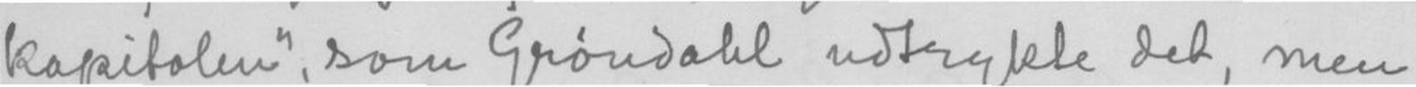kapitalen, som Grøndahl udtrykte det, men
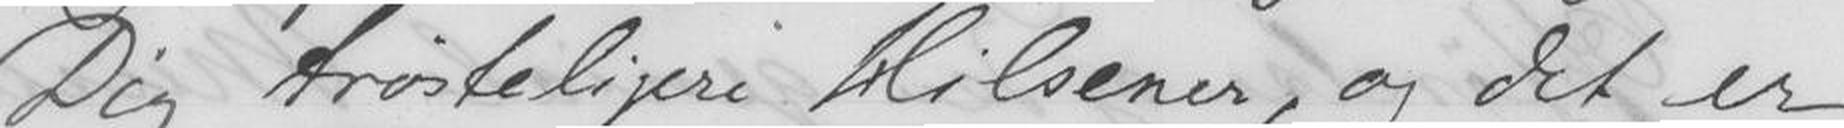Dig trøsteligere Hilsener, og det er
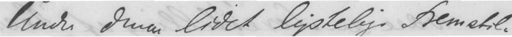Under denne lidet lystelige Fremstil-
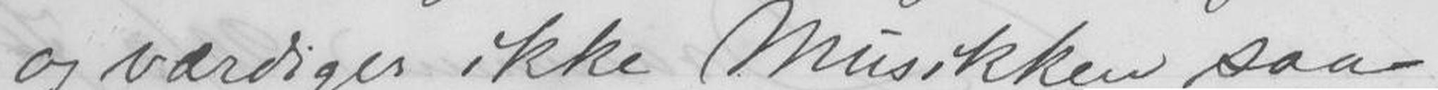og værdiger ikke Musikken saa
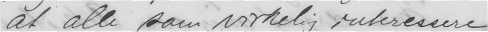at alle som virkelig interessere
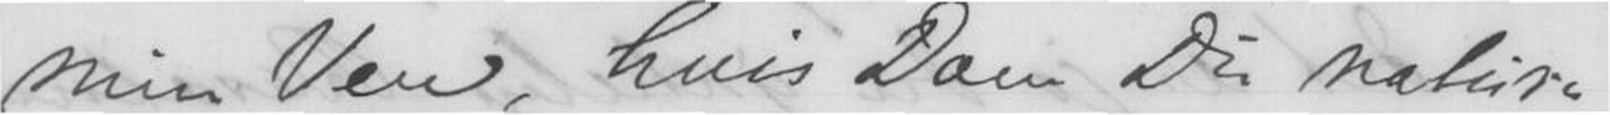min Ven, hvis Dom Du naturligvis
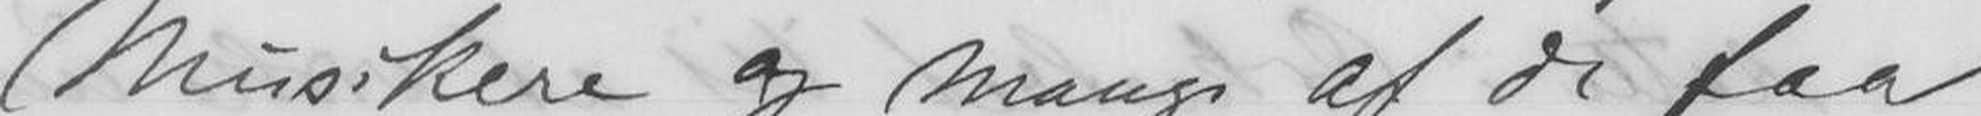Musikere og mange af de faa
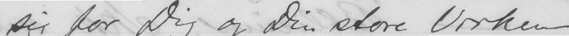sig for Dig og Din store Virken
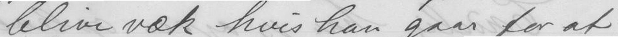blive væk hvis han gaar for at
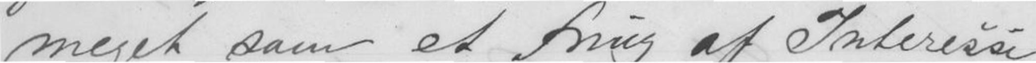meget som et Fnug af Interesse
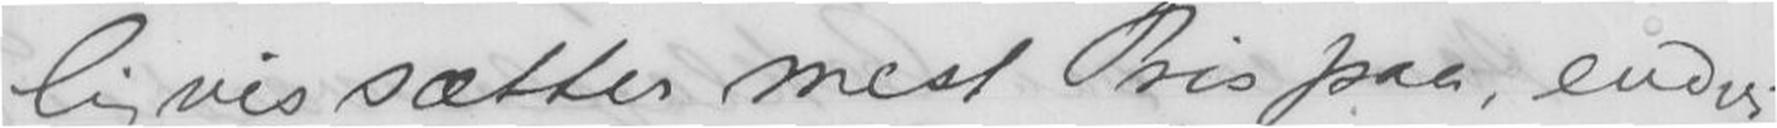sætter mest Pris paa, end-
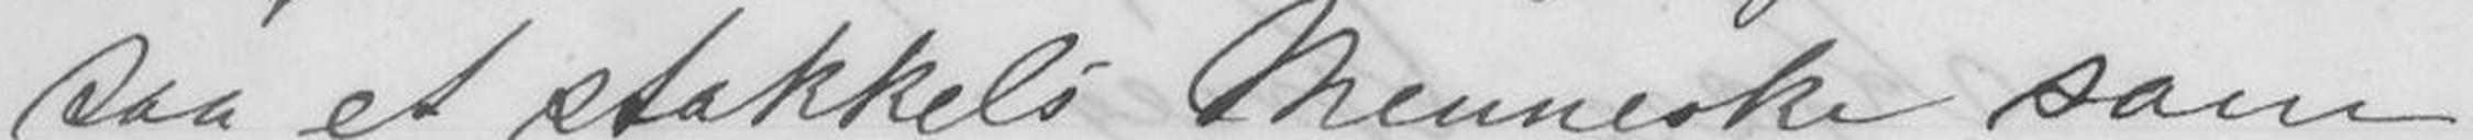saa et stakkels Menneske som
